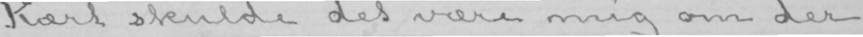Kært skulde det være mig om der
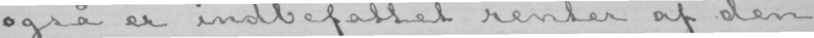også er indbefattet renter af den
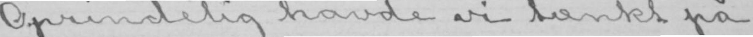Oprindelig havde vi tænkt på
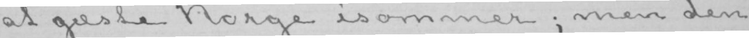at gæste Norge isommer; men den
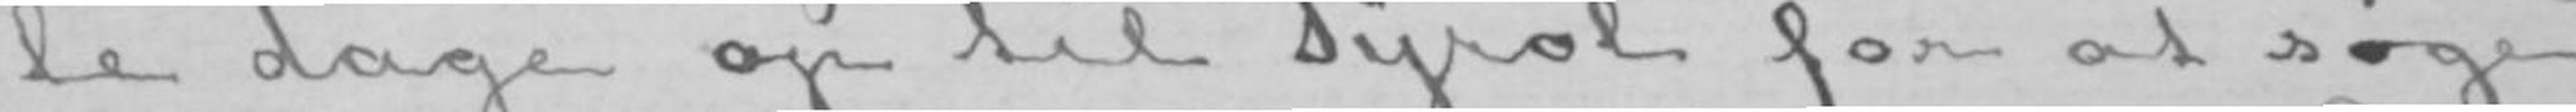le dage op til Tyrol for at søge
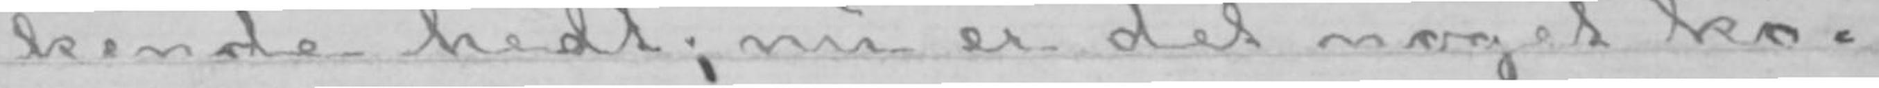kende hedt; nu er det noget kø-
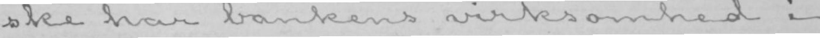ske har bankens virksomhed i
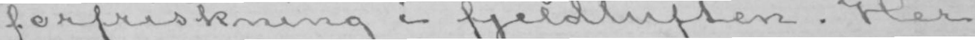forfriskning i fjeldluften. Her
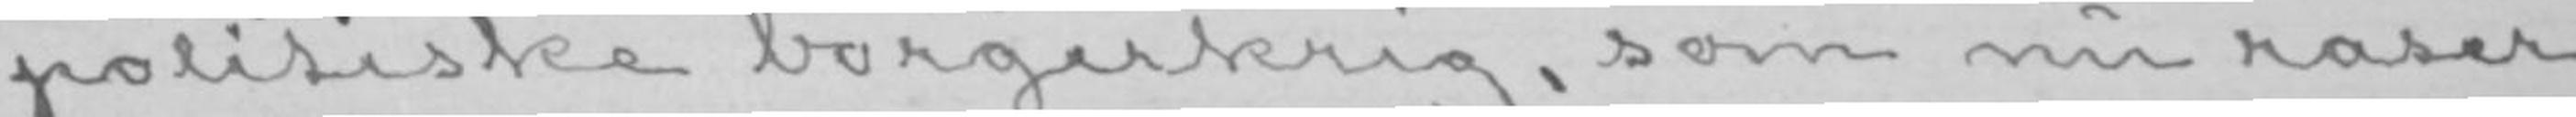politiske borgerkrig, som nu raser
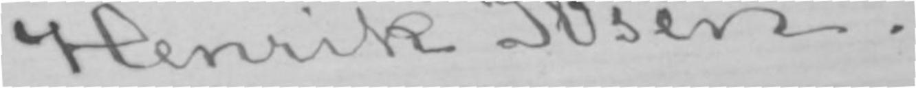Henrik Ibsen.
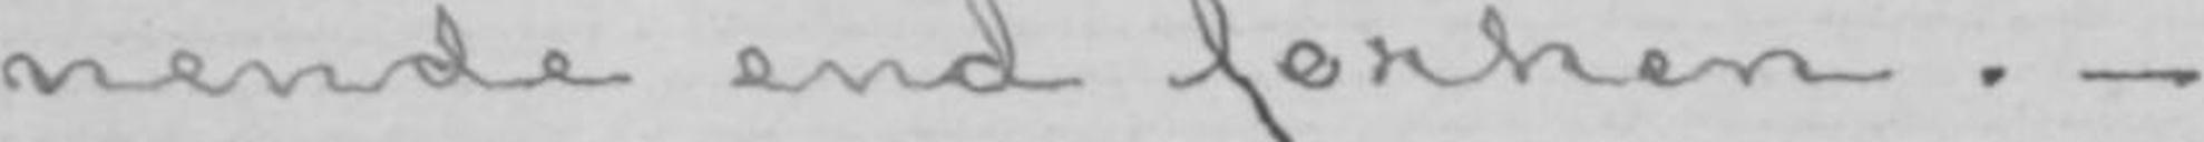nende end forhen.-
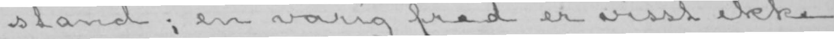stand; en varig fred er visst ikke
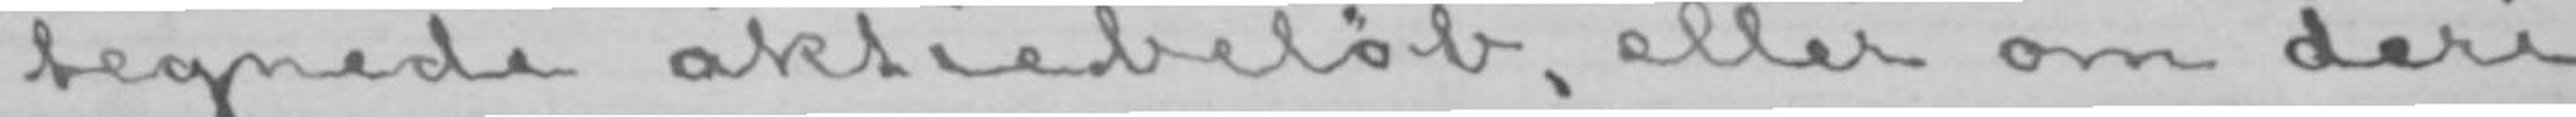tegnede aktiebeløb, eller om deri
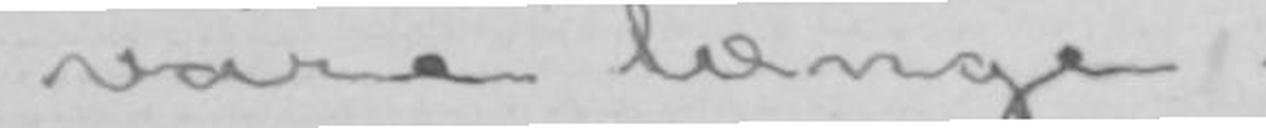vare længe.
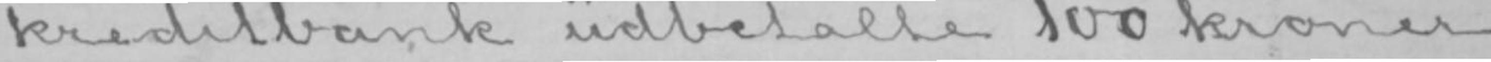kreditbank udbetalte 100 kroner
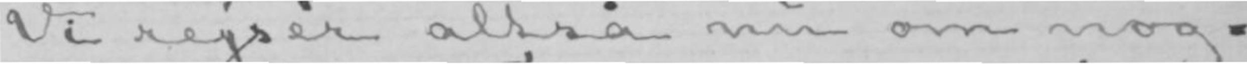Vi rejser altså nu om nog-
